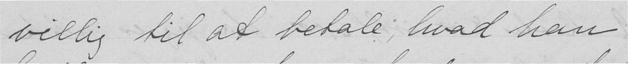villig til at betale, hvad han
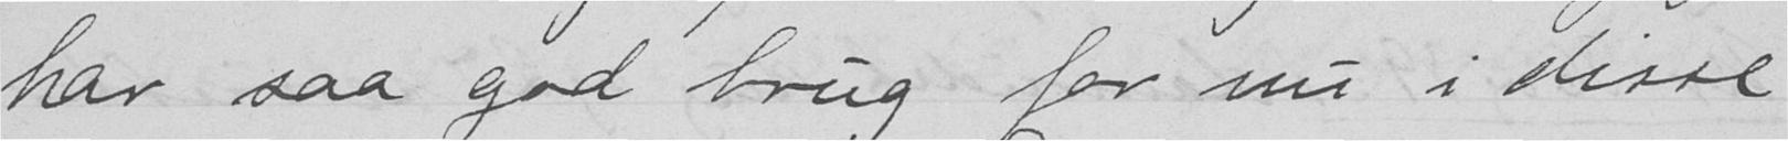har saa god brug for nu i disse
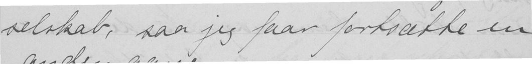selskab, saa jeg faar fortsætte en
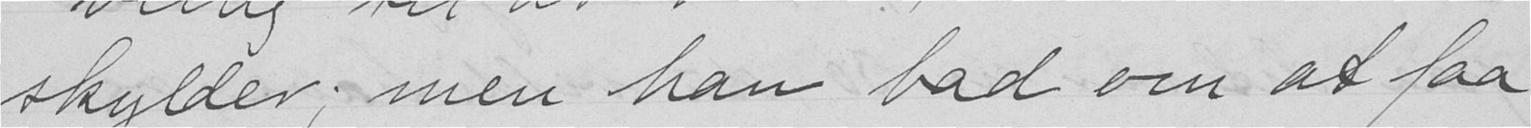skylder; men han bad om at faa
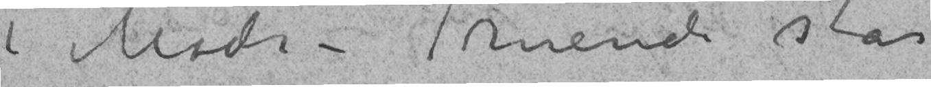i møde – Truende står
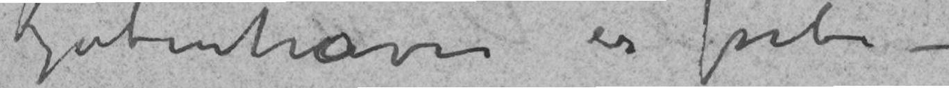Kjøbenhavn er forbi –
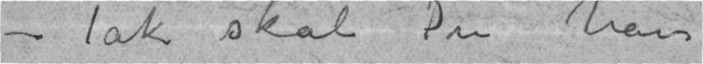– Tak skal Du have
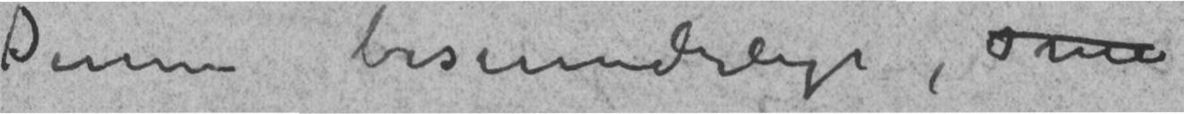Denne besynderlige,
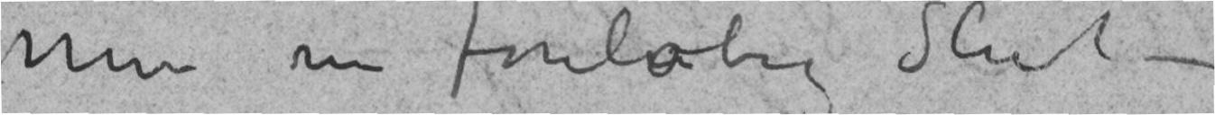Men nu foreløpig Slut –
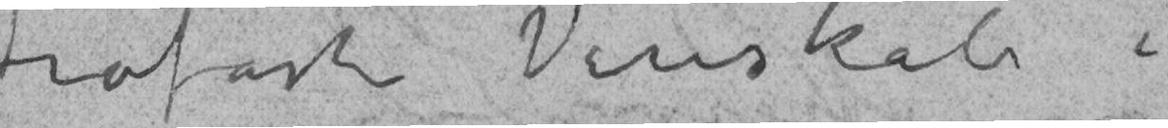trofaste Venskab i
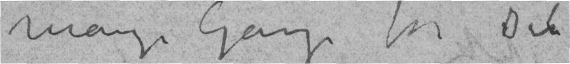mange Gange for Dit
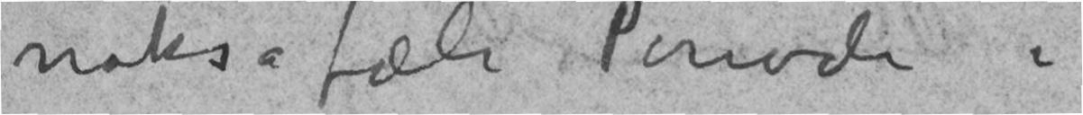nokså fæle Periode i
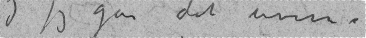og jeg går det uvisse
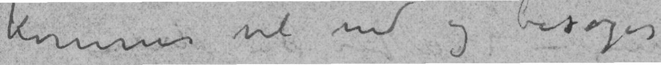kommer vel ned og besøger
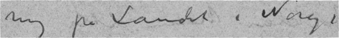mig på Landet i Norge
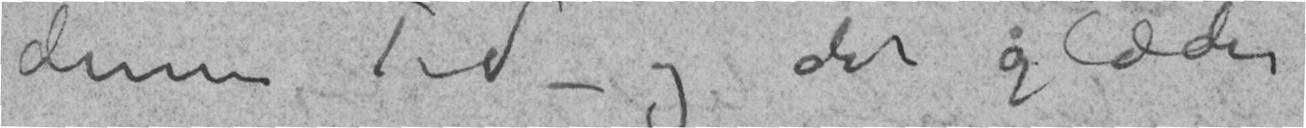denne Tid – og det glæder
